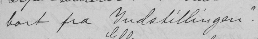bort fra Indstillingen".
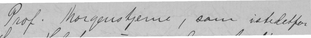Prof. Morgenstjerne, som istedetfor
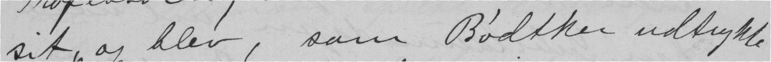sit, og blev, som Bødtker udtrykte
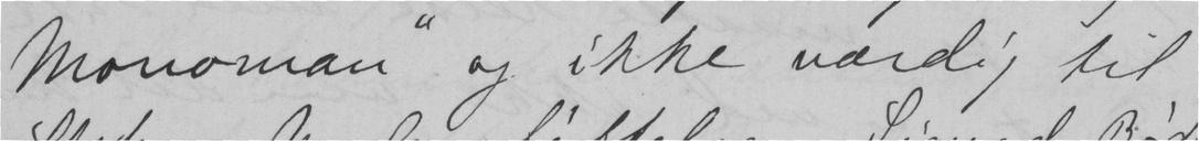Monoman" og ikke værdig til
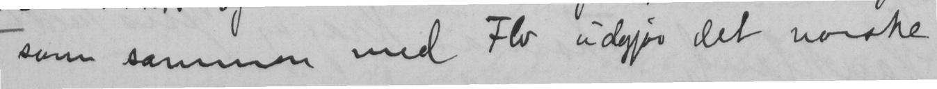som sammen med Flo udgjør det norske
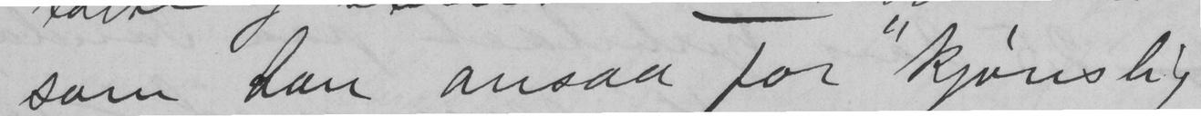som han ansaa for "kjønslig
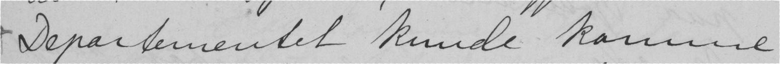Departementet kunde komme
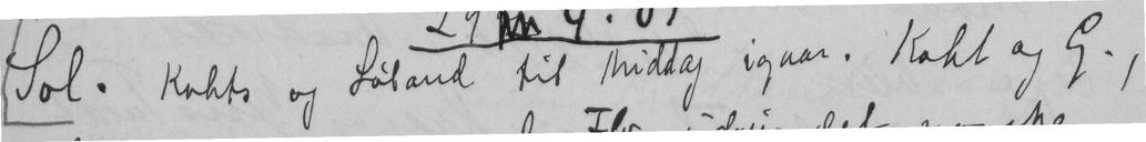Sol. Kohts og Løland til Middag igaar. Koht og G.,
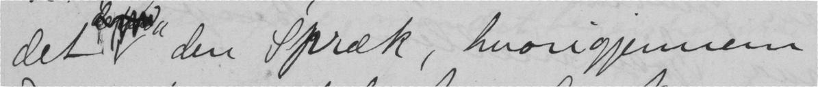det  den Spræk, hvorigjennem
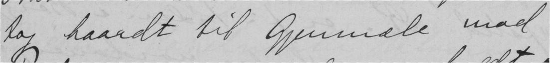tog haardt til Gjenmæle mod
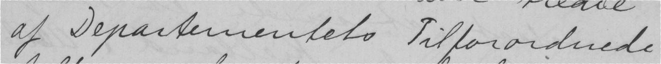af Departementets Tilforordnede
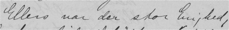Ellers var der stor Enighed,
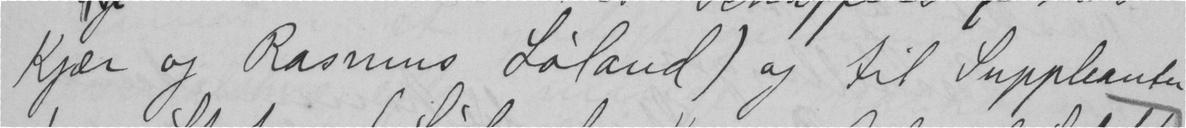Kjær og Rasmus Løland) og til Suppleanter
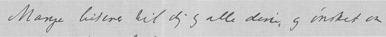Mange hilsener til dig og alle dine og ønsket om
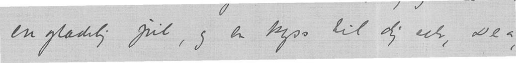en glædelig jul, og en kyss til dig selv, Dea,
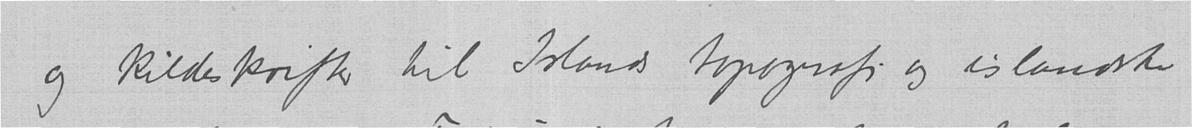og kildeskrifter til Islands topografi og islandske
